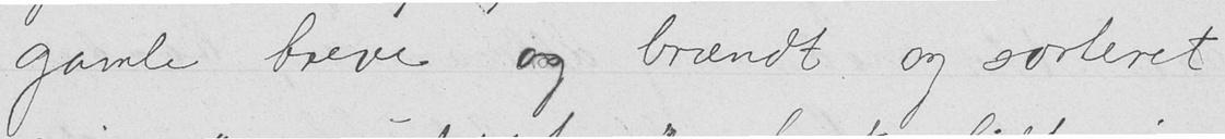gamle breve og brændt og sorteret
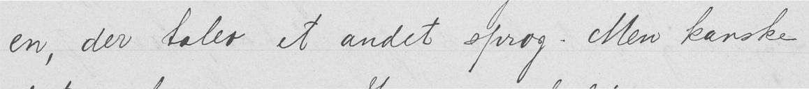en, der taler et andet sprog. Men kanske
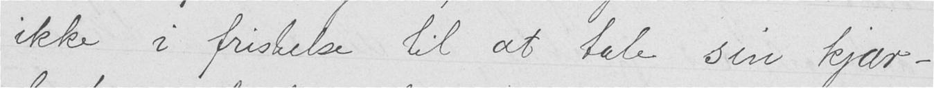ikke i fristelse til at tale sin kjær-
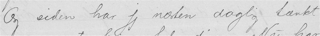Og siden har jeg næsten daglig tænkt
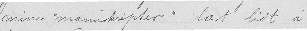mine "manuskripter", læst lidt i
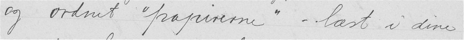og ordnet "papirerne" - læst i dine
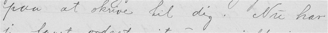paa at skrive til dig. Nu har
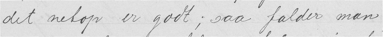det netop er godt; saa falder man
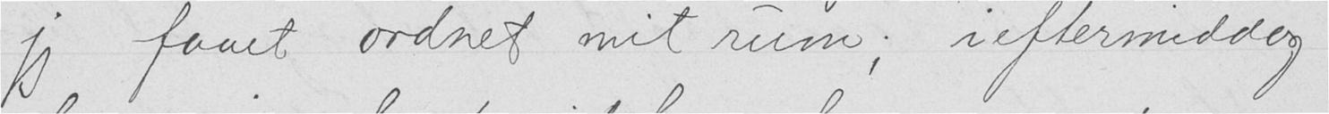jeg faaet ordnet mit rum; ieftermiddag
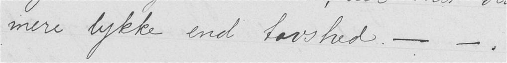mere lykke end tavshed - -.
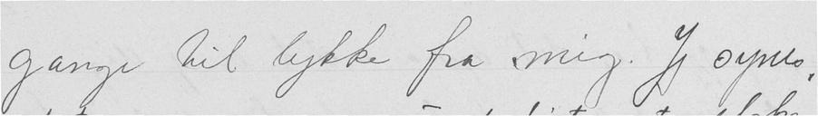gange til lykke fra mig. Jeg synes ,
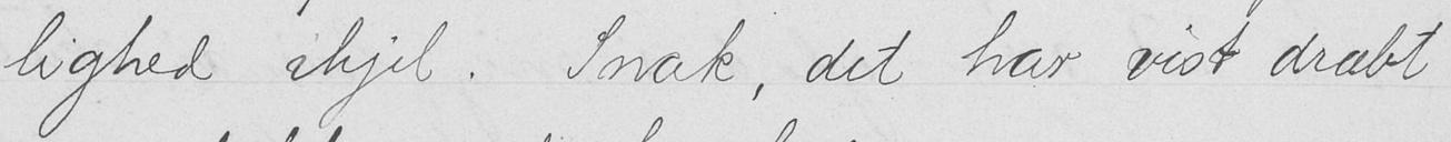lighed ihjel. Snak, det har vist dræbt
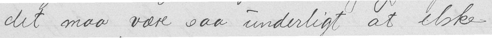det maa være saa underligt at elske
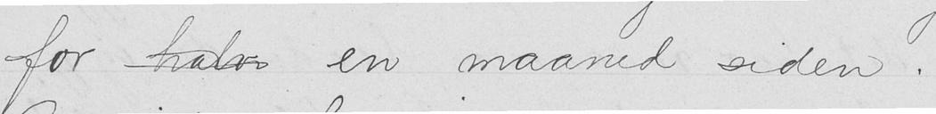for halv en maaned siden.
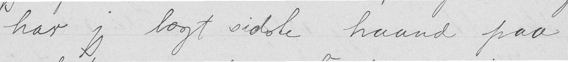har jeg lagt sidste haand paa
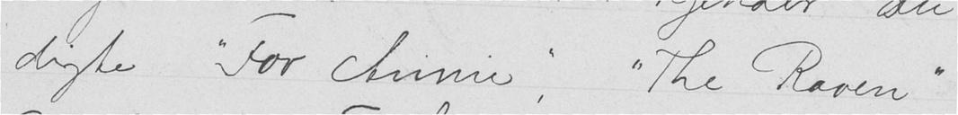digte "For Annie", "The Raven",
